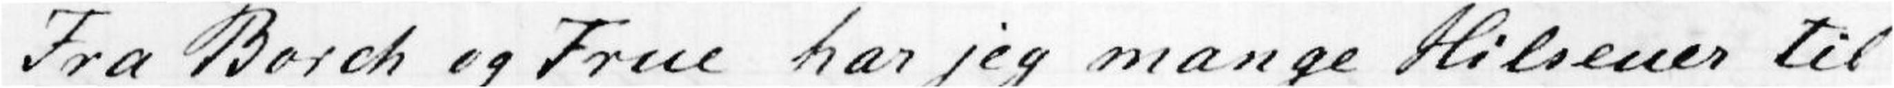Fra Borch og Frue har jeg mange Hilsener til
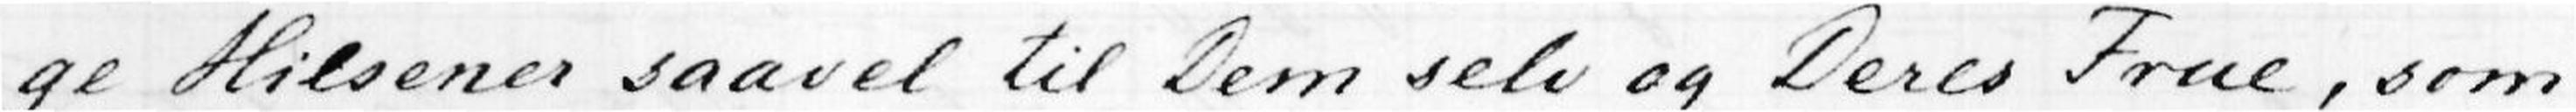ge Hilsener til Dem selv og Deres Frue, som
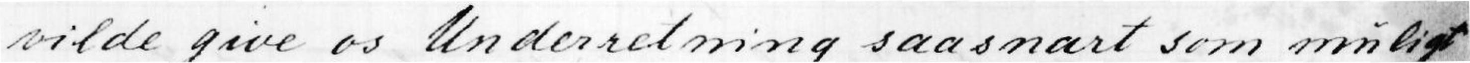vilde give os Underretning saasnart som muligt
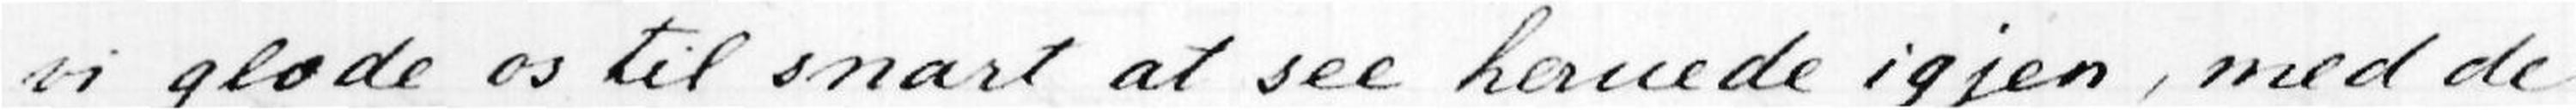vi glæde os til snart at see hernede igjen, med de
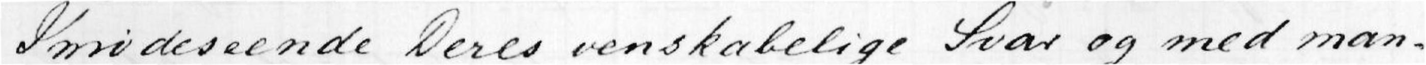Imødeseende Deres venskabelige Svar og med man-
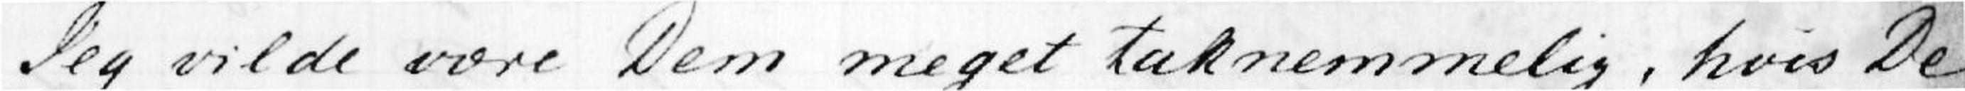Jeg vilde være Dem meget teknemmelig, hvis De
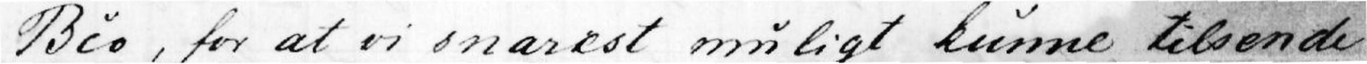Bco, for at vi snarest muligt kunne tilsende
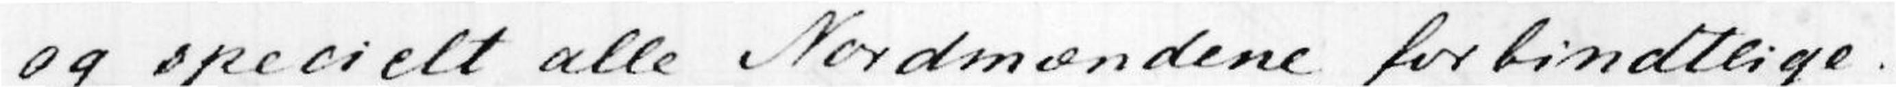og specielt alle Nordmændene forbindlige.
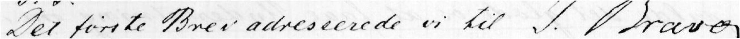Det første Brev adresserede vi tilJ. Bravo.
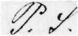P.S.
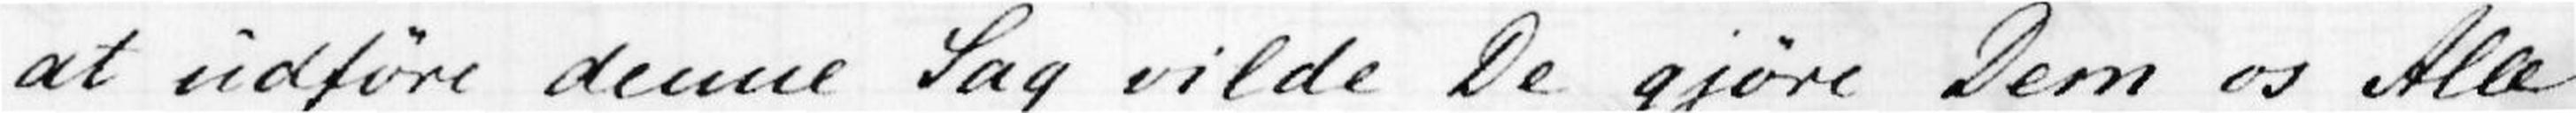at udføre denne Sag vilde De gjøre Dem os Alle
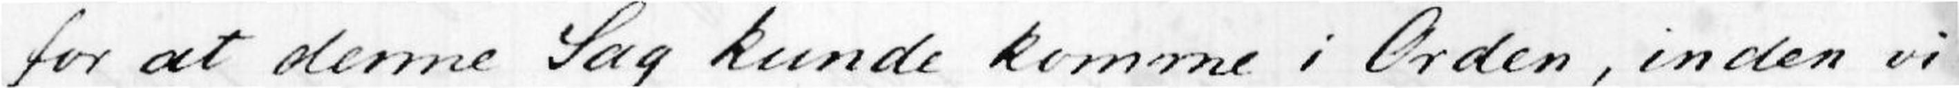for at denne Sag kunde komme i Orden, inden vi
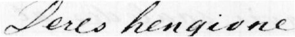Deres hengivne
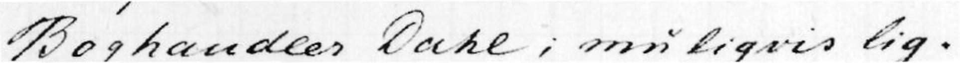Boghandler Dahl; muligvis lig-
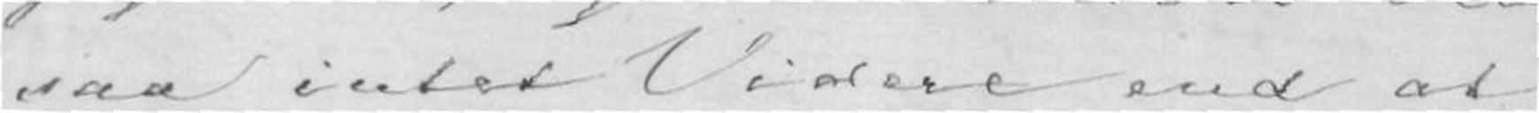saa intet Videre end at
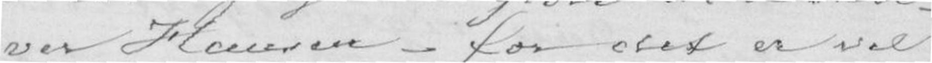ver Hansen - for det er vel
